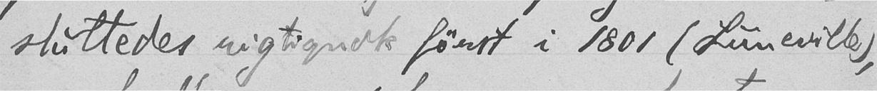sluttedes rigtignok først i 1801 (Luneville),
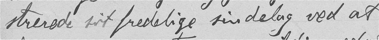strerede sit fredelige sindelag ved at
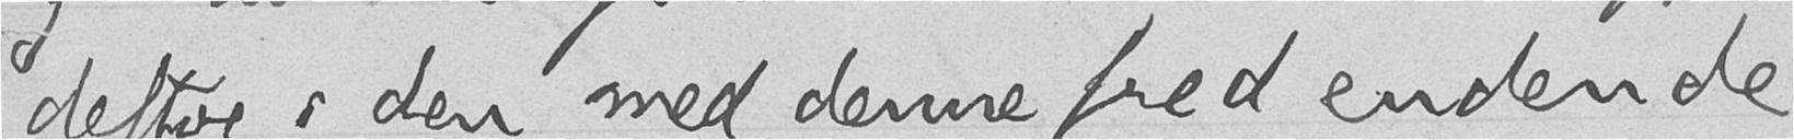deltog i den med denne fred endende
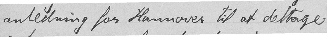anledning for Hannover til at deltage
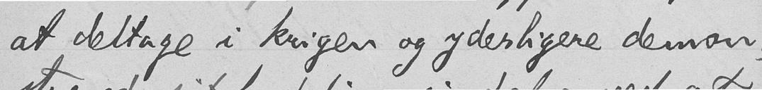at deltage i krigen og yderligere demon-
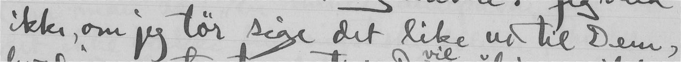ikke, om jeg tør sige det like ud til Dem,
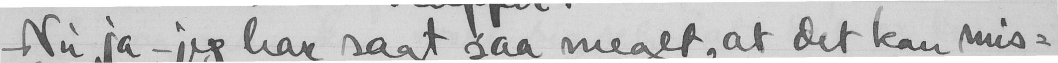Nu sa - jeg har sagt saa meget, at det kan mis-
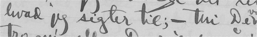hvad jeg segter til; - thi De
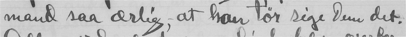mand saa ærlig, at han tør sige Dem det.
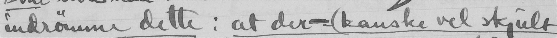indrømme dette : at der (kanske vel skjult
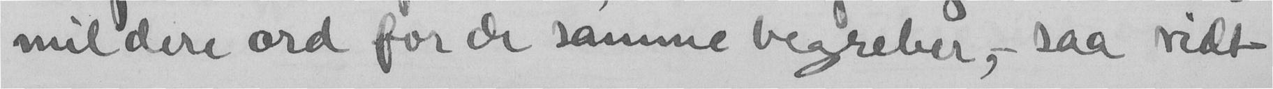mildere ord for de samme begreber, - saa vidt
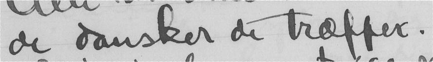de dansker de træffer.
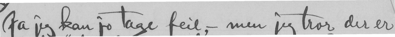Ja jeg kan jo tage feil, - men jeg tror der er
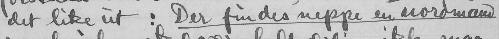det like ut : Der findes neppe en nordmand
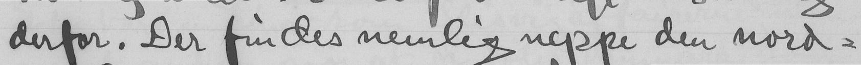derfor. Der findes nemlig neppe den nord-
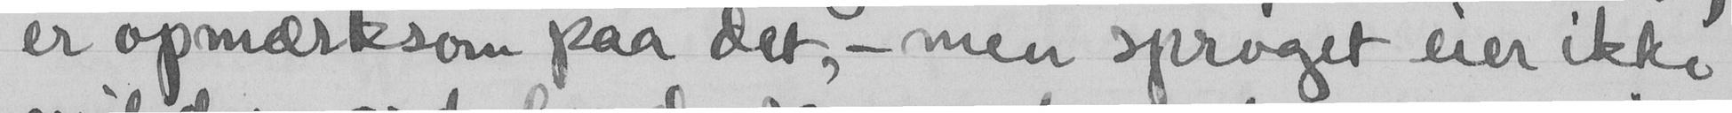er opmærksom paa det, - men sproget eier ikke
